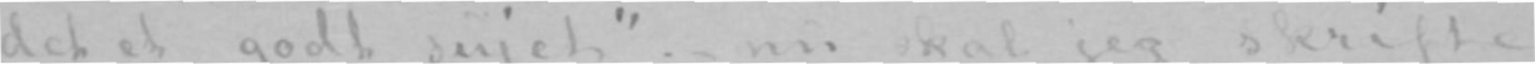det et godt sujet»;- nu skal jeg skrifte
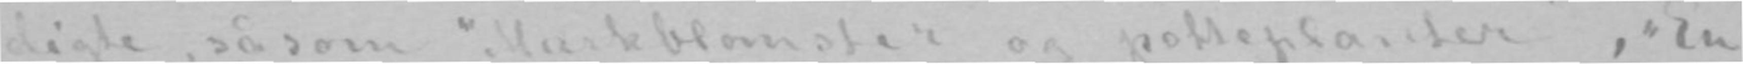digte, såsom «Markblomster og potteplanter», «En
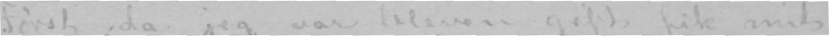Først da jeg var bleven gift fik mit
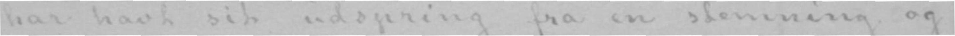har havt sit udspring fra en stemning og
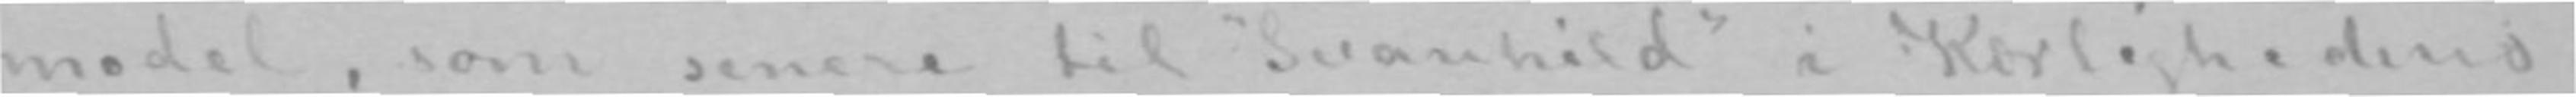model, som senere til «Svanhild» i «Kærlighedens
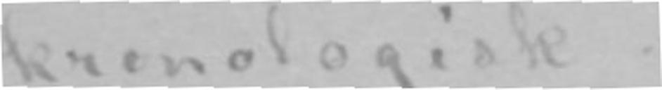kronologisk.
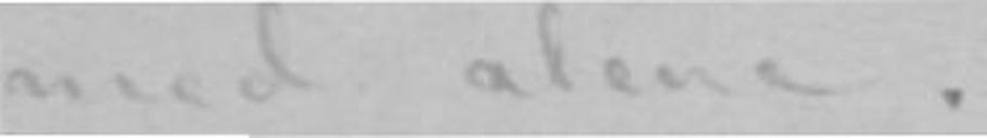med alene.
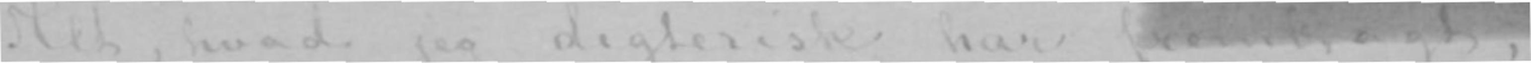Alt, hvad jeg digterisk har frembragt,
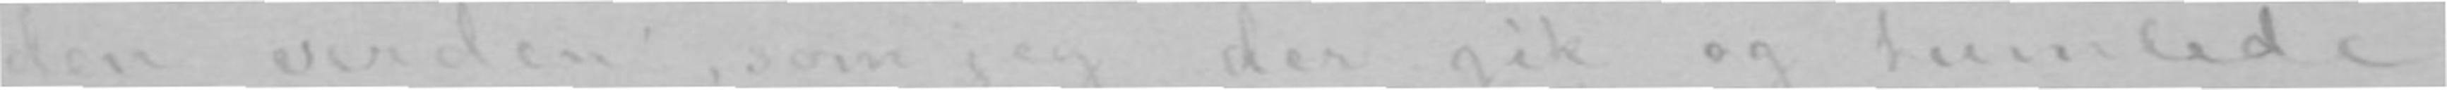den verden, som jeg der gik og tumlede
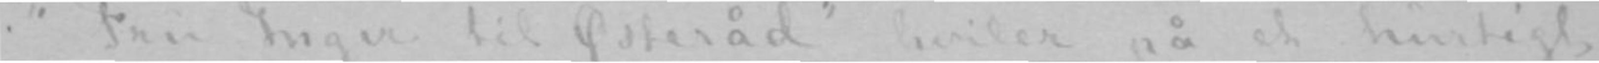«Fru Inger til Østeråd» hviler på et hurtigt
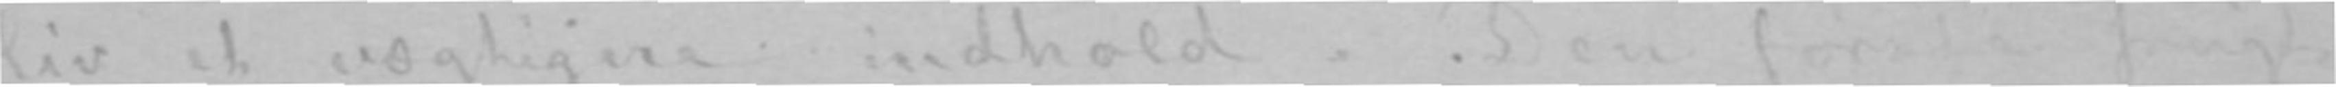liv et vægtigere indhold. Den første frugt
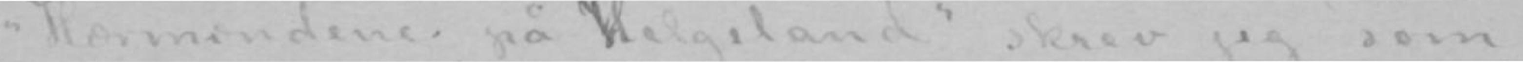«Hærmændene på Helgeland» skrev jeg som
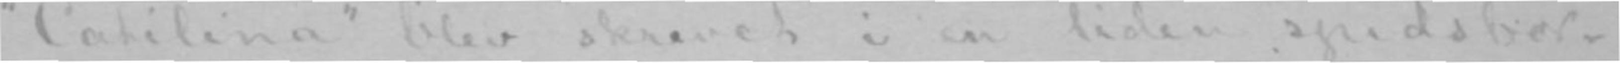«Catilina» blev skrevet i en liden spidsbor-
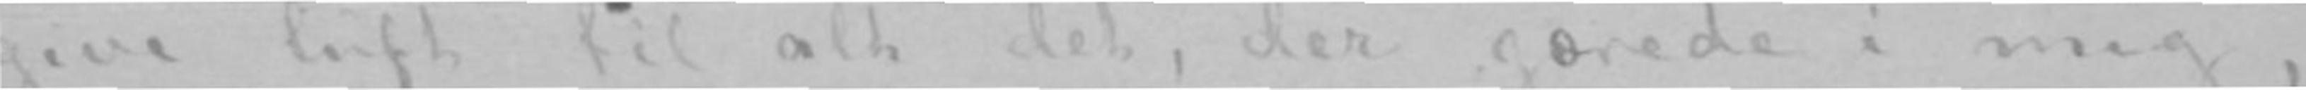give luft til alt det, der gærede i mig,
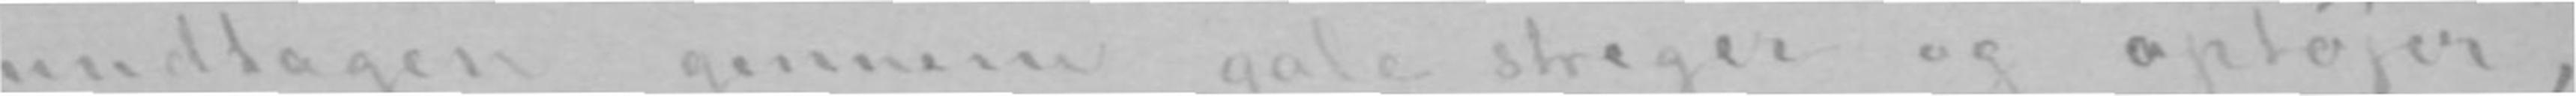undtagen gennem gale streger og optøjer,
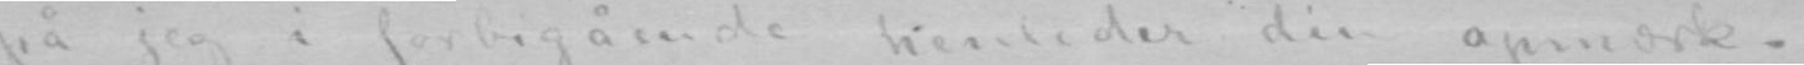på jeg i forbigående henleder din opmærk-
